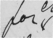for
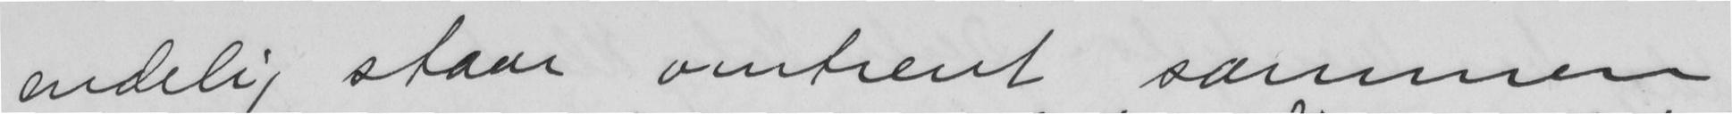endelig staar omtrent sammen
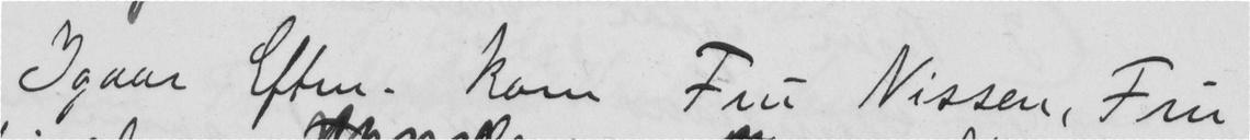Igaar Eften. kom Fru Nissen, Fru
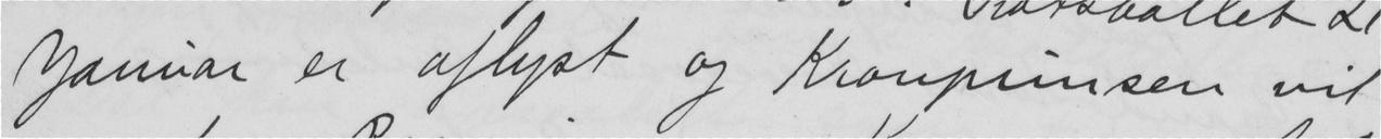Januar er aflyst og Kronprinsen vil
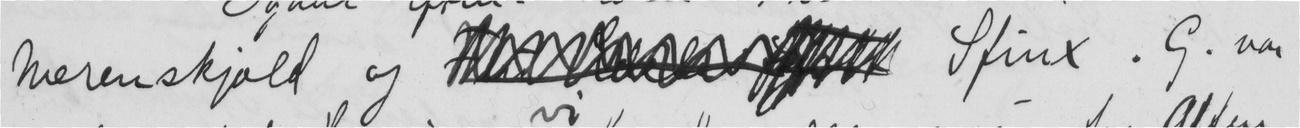Werenskjold og  Sfinx. G. var
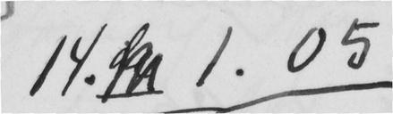14. 1.05
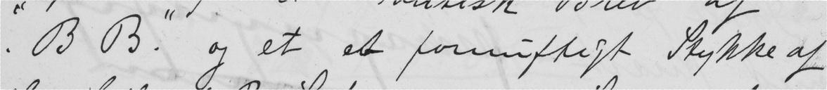"B B." og et et fornuftigt Stykke af
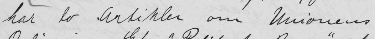har to Artikler om Unionens
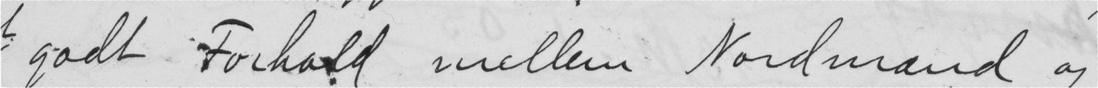godt Forhold mellem Nordmænd og
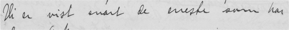Vi er vist snart de eneste som har
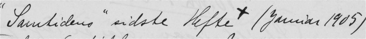"Samtidens" sidste Hefte (Januar 1905)
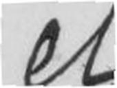et
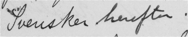Svensker herefter.
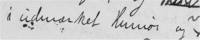i udmærket Humør og
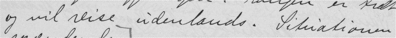og vil reise udenlands. Situationen
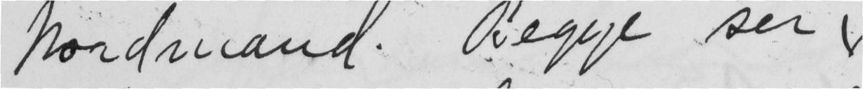Nordmand. Begge ser
